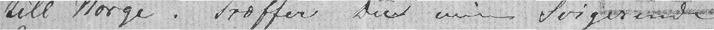till Norge. Træffer Du min Svigerinde
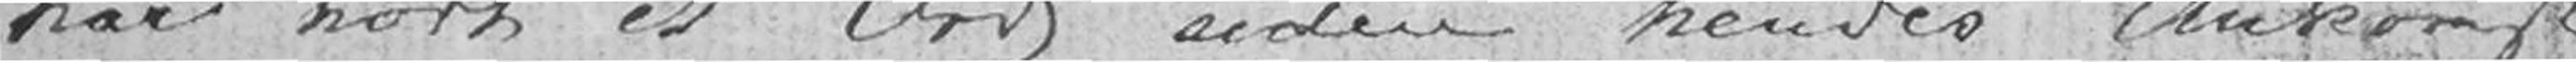har hørt et Ord siden hendes Ankomst
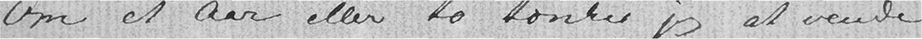Om et Aar eller to tænker jeg at vende
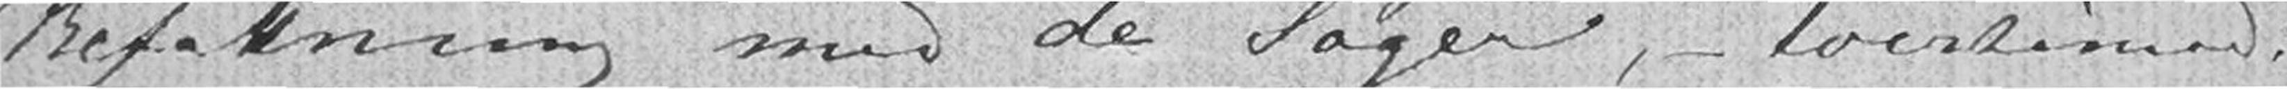Befattning med de Sager,- tvertimod!
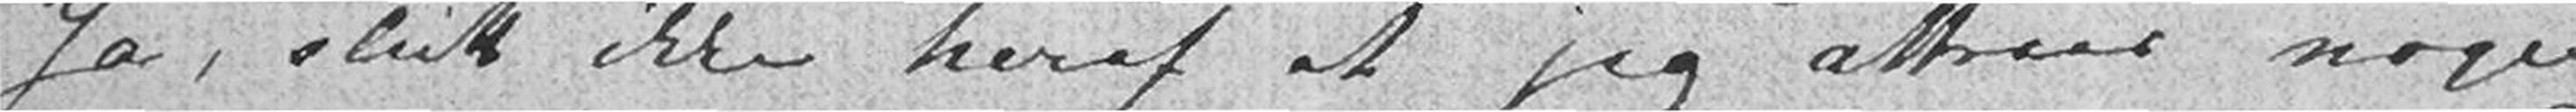Ja, slutt ikke heraf at jeg attraar nogen
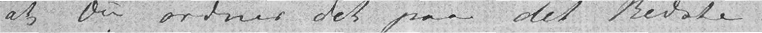at Du ordner det paa det Bedste.
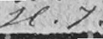H. I.
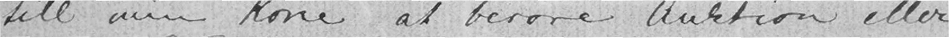till min Kone at berøre Auktion eller
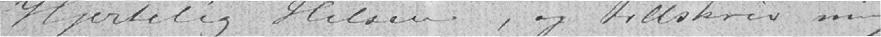Hjertelig Hilsen, og tillskriv mig
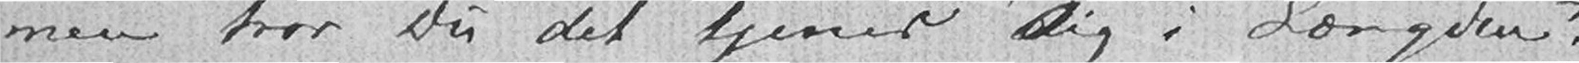men tror Du det tjener Dig i Længden?
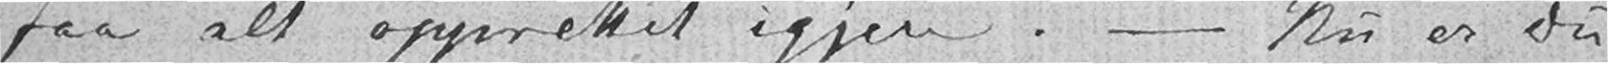faa alt opprettet igjen.- Nu er Du
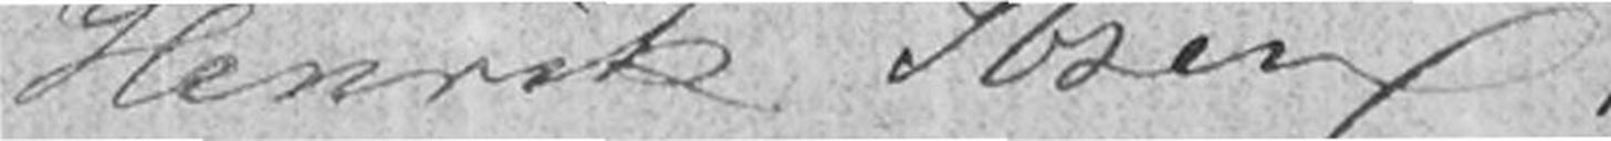Henrik Ibsen.
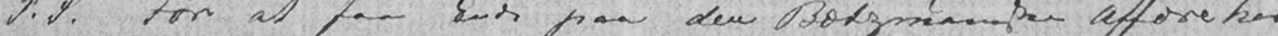P. S. For at faa Ende paa den Bætzmannske Affære
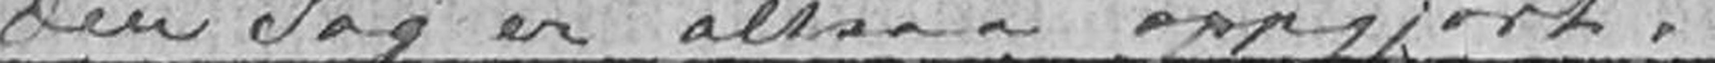Den Sag er altsaa oppgjort.
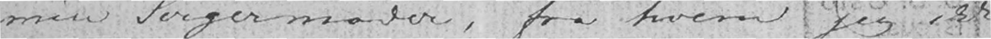min Svigermoder, fra hvem jeg ikke
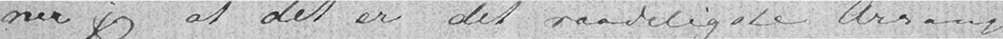ner jeg at det er det raadeligste Arrange-
